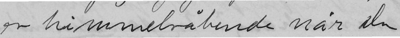er himmelråbende når den
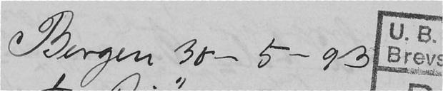Bergen 30 - 5 - 93
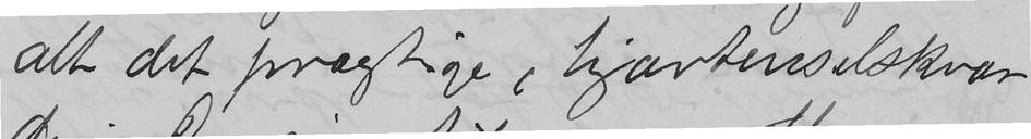alt det prægtige, hjærtenselskvær-
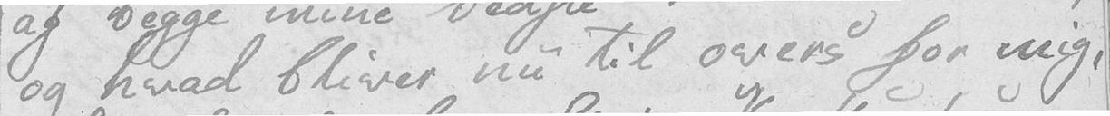og hvad bliver nu til overs for mig,
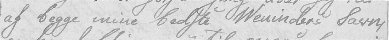af begge mine bedste Veninders Savn,
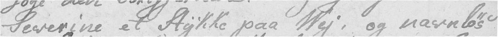Severine et Stykke paa Vej, og navnløs
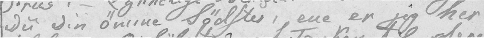Du Din ømme Sødster, ene er jeg her
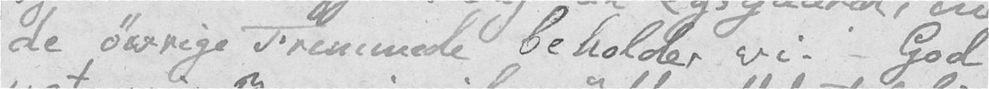de øvrige Fremmede beholder vi. – God
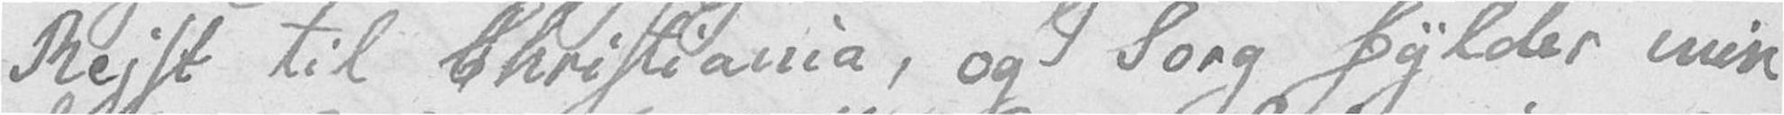Rejst til Christiania, og Sorg fylder min
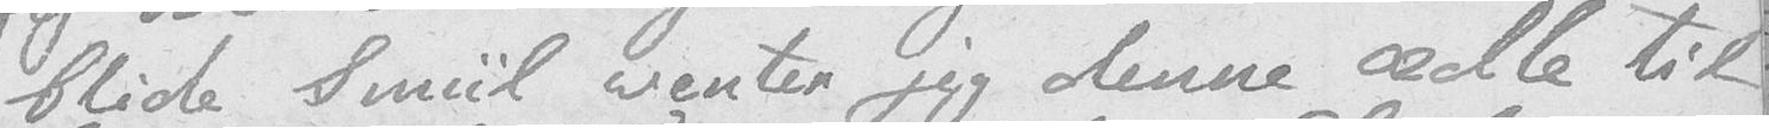blide Smiil venter jeg denne ædle til
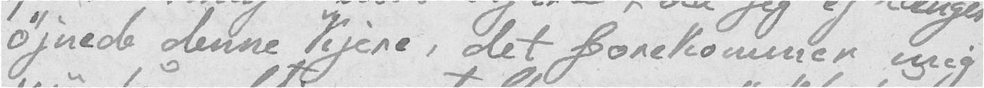øjnede denne Kjere, det forekommer mig
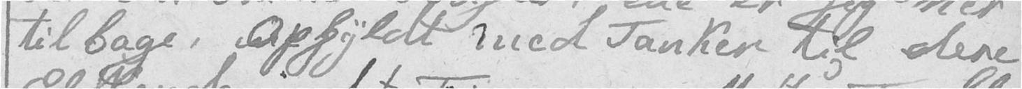tilbage. Opfyldt med Tanker til dere
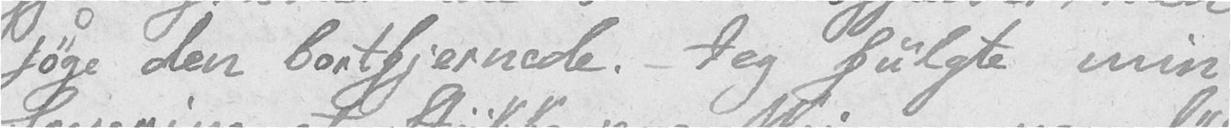søge den bortfjernede. – Jeg fulgte min
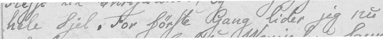hele Sjel. For første Gang lider jeg nu
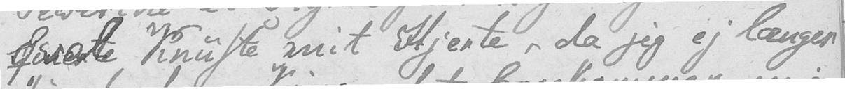smerte knuste mit Hjerte, da jeg ej længer
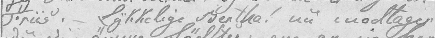Friis. – Lykkelige Bertha! nu modtager
